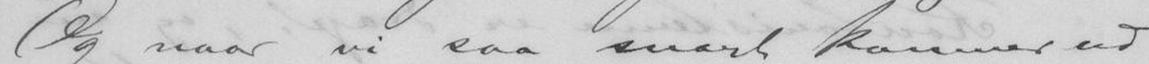Og naar vi saa snart kommer ud
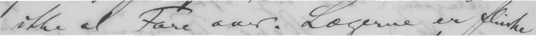ikke al Fare over. Lægerne er flinke
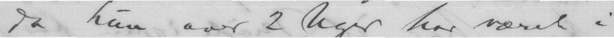da hun over 2 Uger har været i
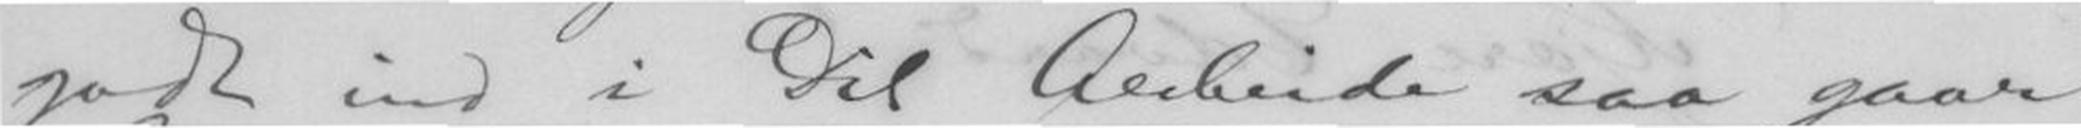godt ind i Dit Arbeide saa gaar
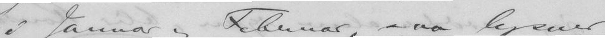i Januar og Februar, saa lysner
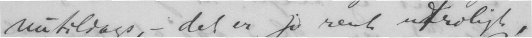nutildags, - det er jo rent utroligt,
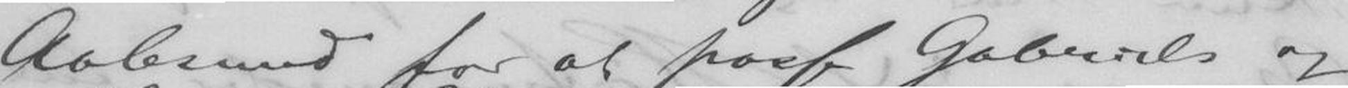Aalesund for at passe Gabriels og
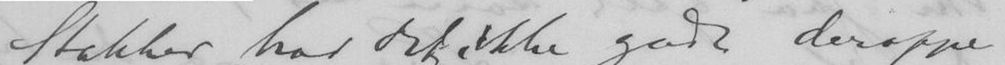Stakker, har det ikke godt deroppe.
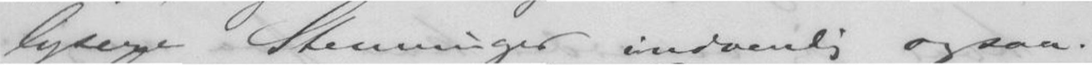lysere Stemninger indvendig ogsaa.
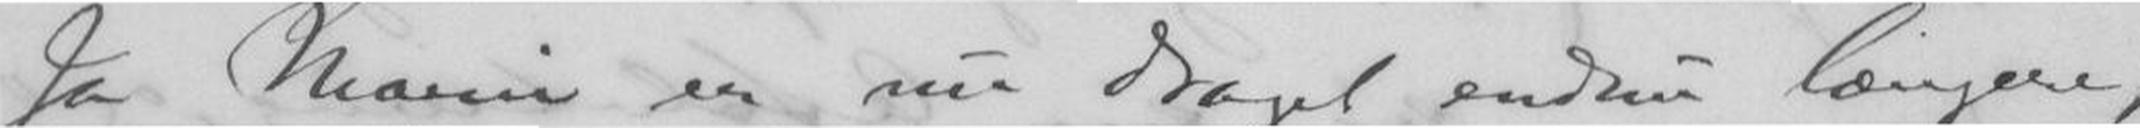Ja Marie er nu draget endnu længere,
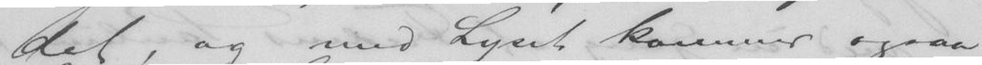det, og med Lyset kommer ogsaa
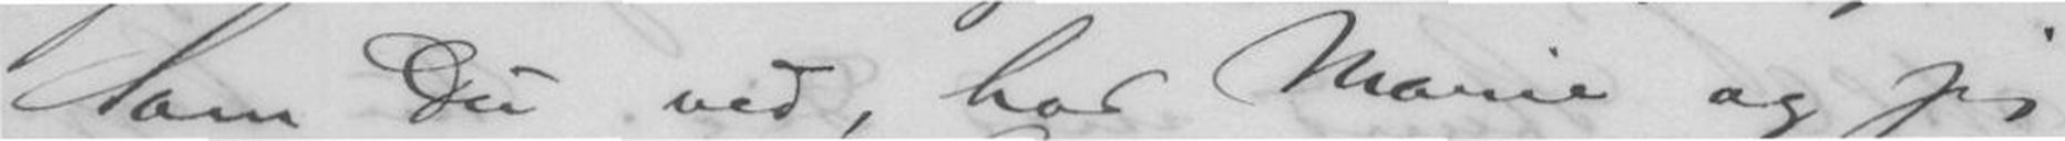Som Du ved, har Marie og jeg
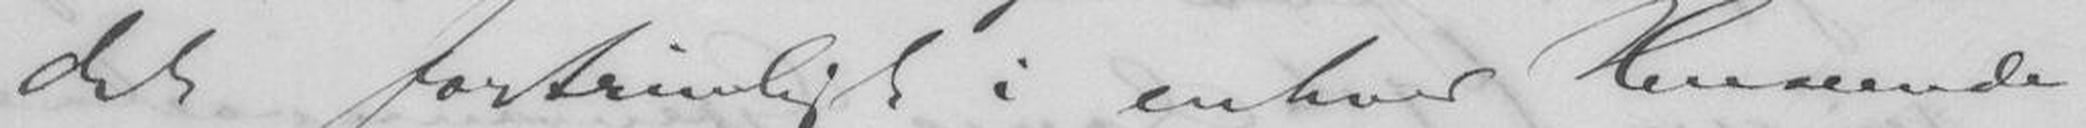det fortrinligt i enhver Henseende.
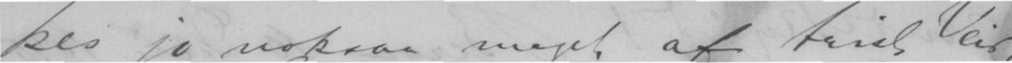kes jo noksaa meget af trist Veir,
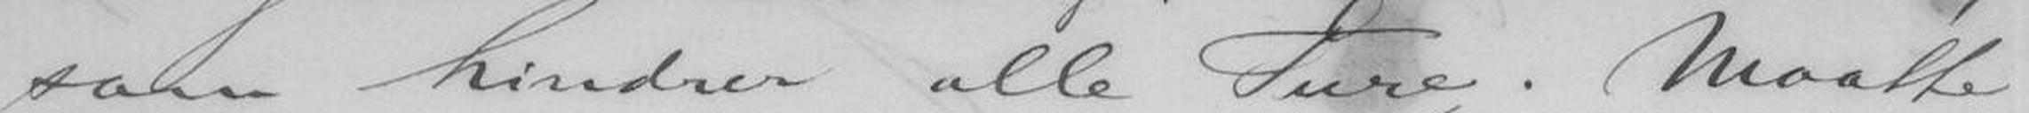som hindrer alle Ture. Maatte
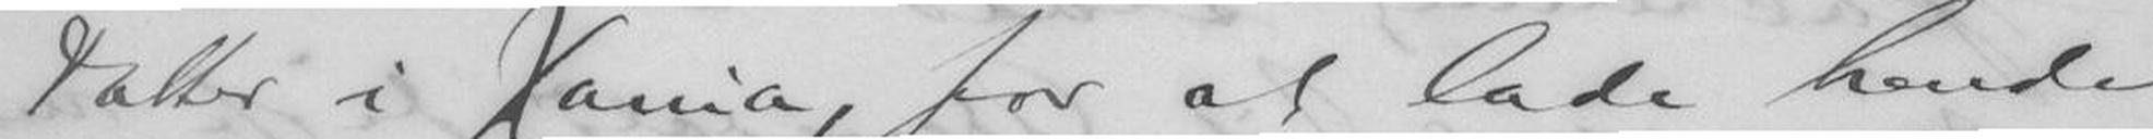Datter i Xania, for at lade hende
